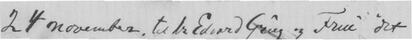24 november til hr Edvard Grieg og Frue det
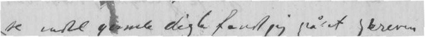se endel gamle digte fandt jeg på et skreven
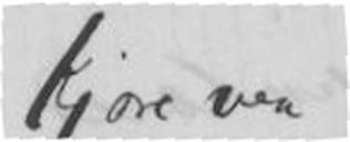Kjære Ven!
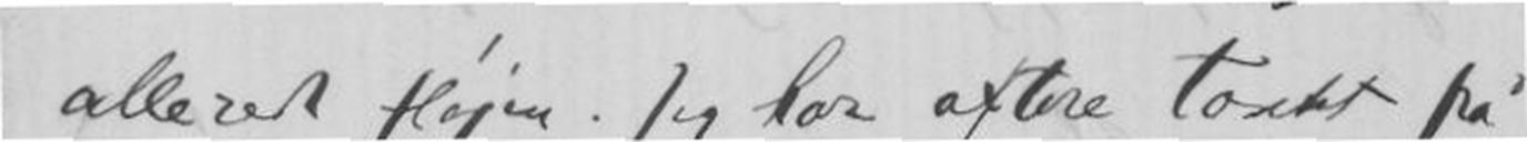allerede fløjet. Jeg har tænkt på
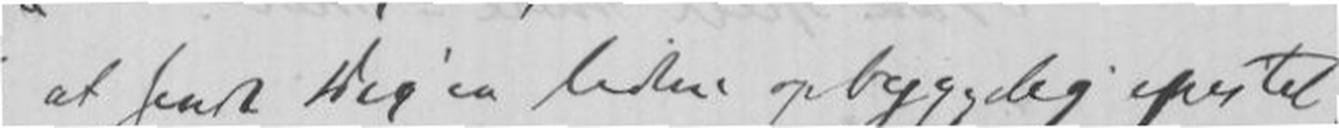at sende Dig en liten opbyggelig epistel,
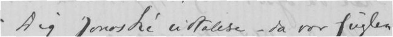Dig Jonas Lie udtalse - da var fuglen
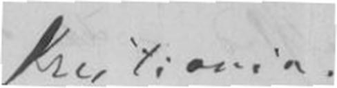Kristiania
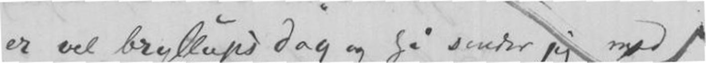er vel bryllupsdag og så sender jeg med
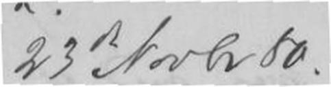23de Novbr 80.
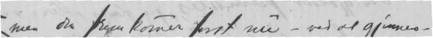men den fremkomer først nu - ved at gjennes-
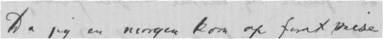Da jeg en morgen kom op forat prise
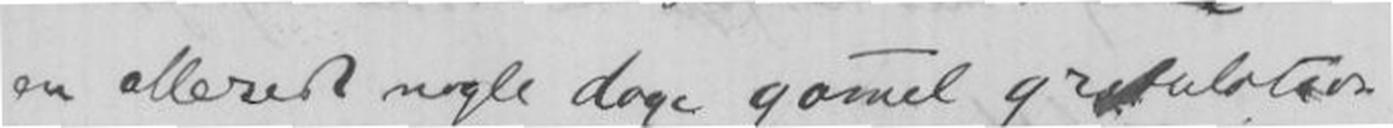en allerede nogle dage gamel gratulation
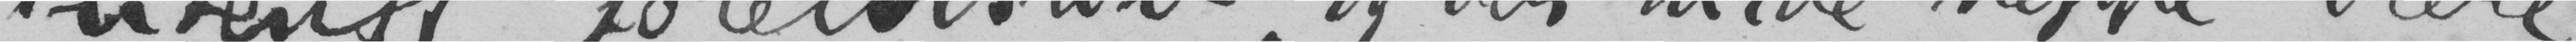intenst følelsesliv, og der turde neppe være
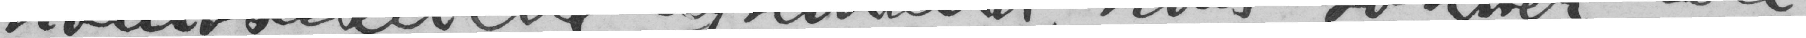homosexuelle ægtemænd, hvis sønner ere
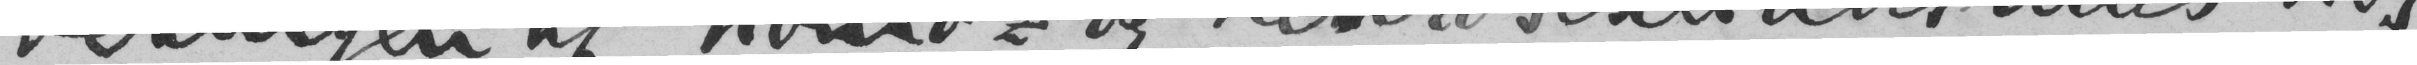vexlingen af homo- og heterosexualismus hos
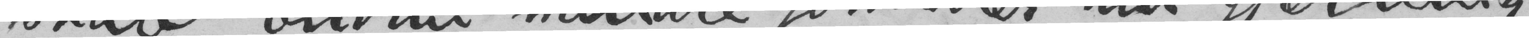skab. Endnu mindre forklarer hin gjætning
